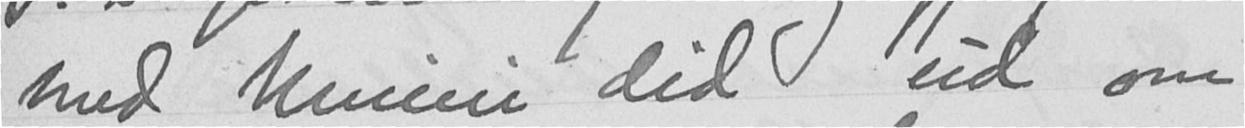med Minni did ud om
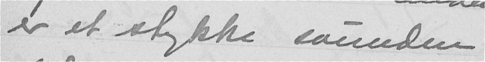er et stykke svunden
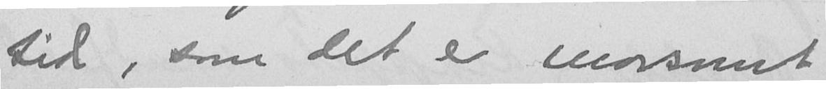tid, som det er morsomt
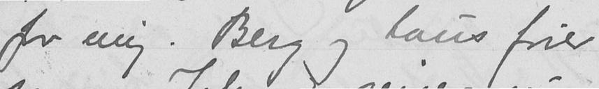for mig. Berg og Lous fører
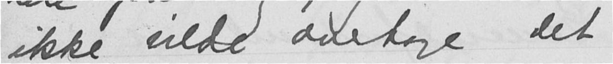ikke vilde overtage det
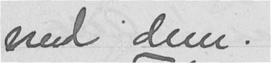med dem.
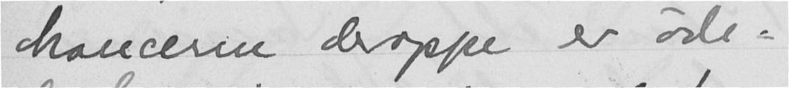chancerne deroppe er øde-
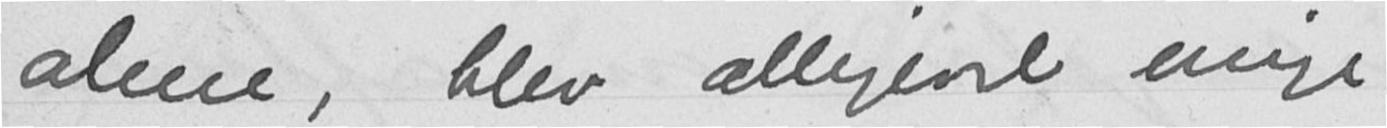alene, blev alligevel enige
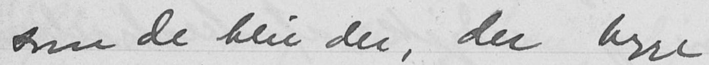som de blir der, der begge
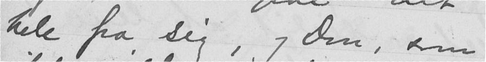hele fra sig, og Don, som
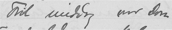Til middag var Don
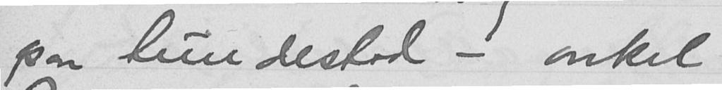paa Lundestad - onkel
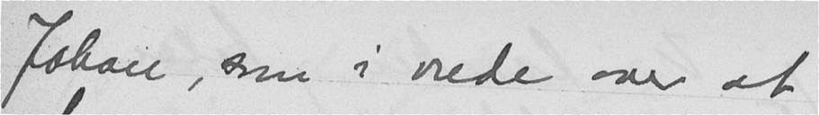Johan, som i vrede over at
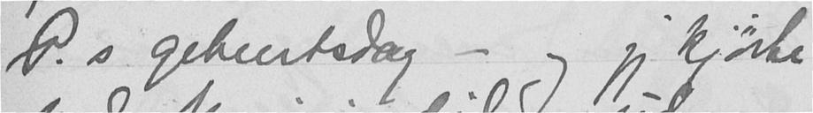P.s geburtsdag - og jeg kjørte
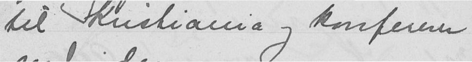til Kristiania og konfererer
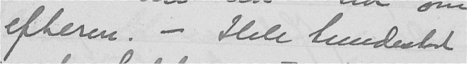efterm. - Hele Lundestad
